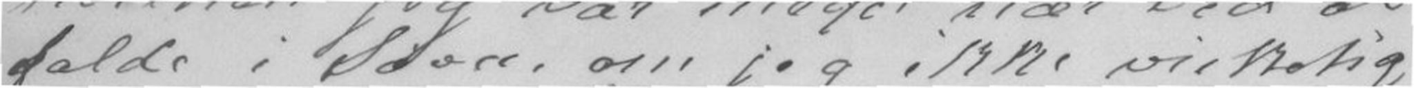falde i Søvn, om jeg ikke virkelig
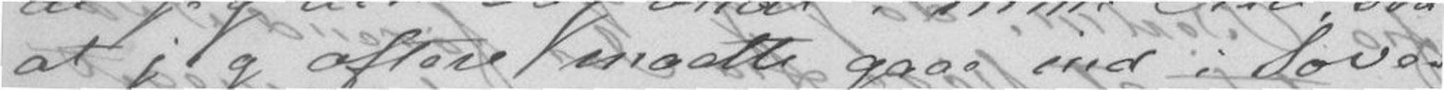at jeg oftere maatte gaae ind i Sove-
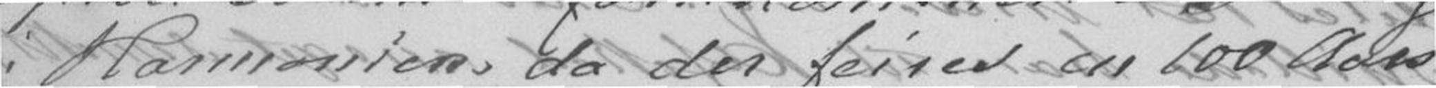i Harmonien da der feires en 100 Aars
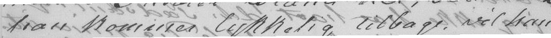han kommer lykkelig tilbage, vil han
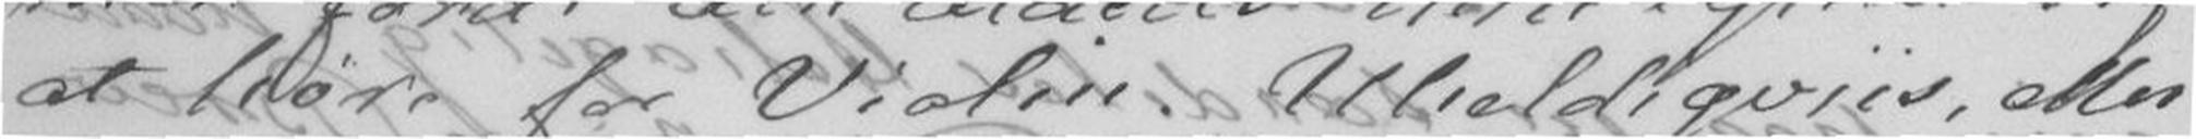at høre for Violin. Uheldigviis, eller
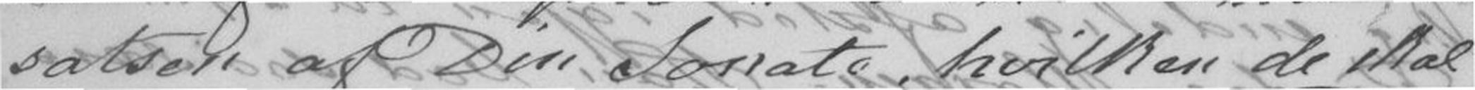satsen af Din Sonate hviken de skal
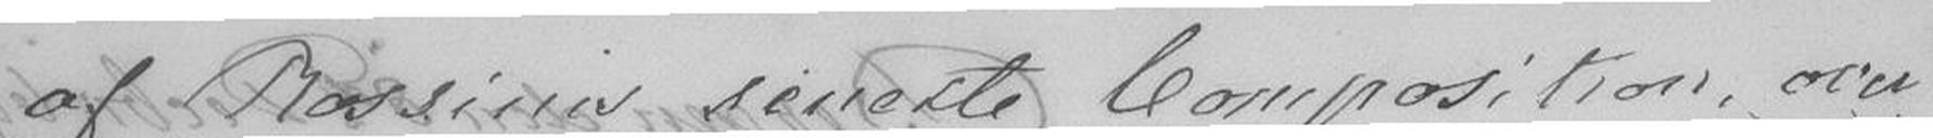af Rossinis seneste Composition, over
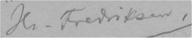Hr. Fredriksen,
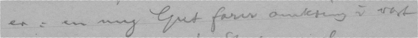er: en ung Gut farer omkring i vort
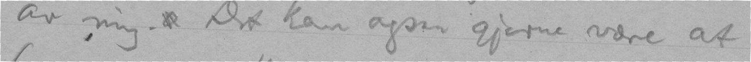av mig. o Det kan ogsaa gjerne være at
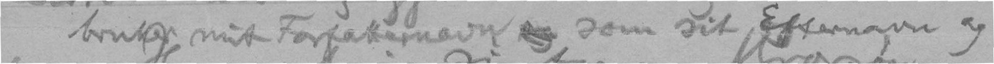bruker mit Forfatternavn og som sit Efternavn og
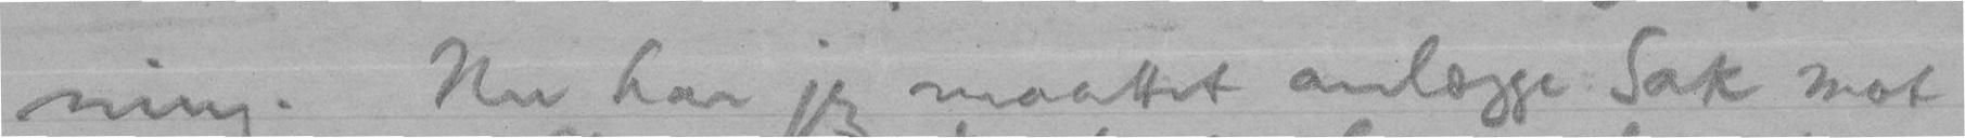ning. Nu har jeg maattet anlægge Sak mot
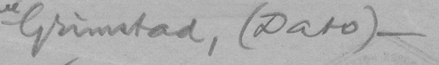Grimstad, (Dato) -
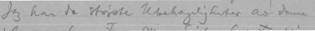Jeg har de største Ubehageligheter av denne
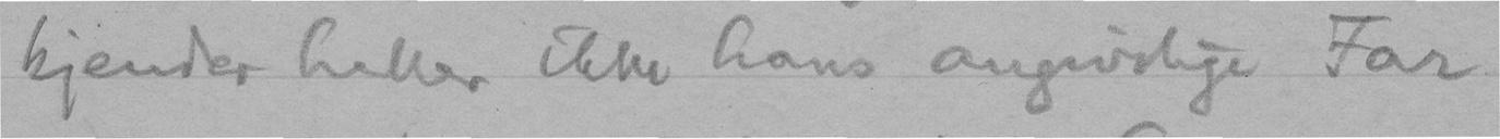kjender heller ikke hans angivelige Far
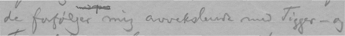de forfølger mig avvekslende med Tigger - og
